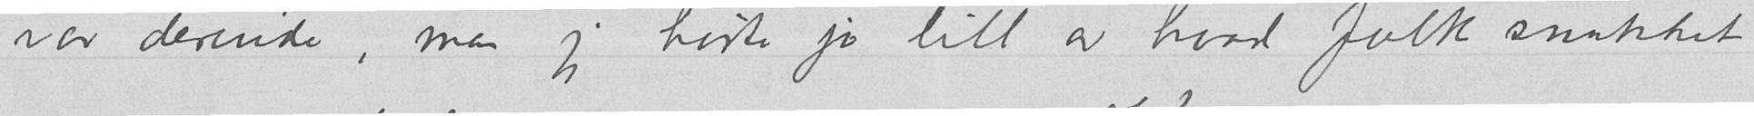var derinde, men jeg hørte jo litt av hvad folk snakket
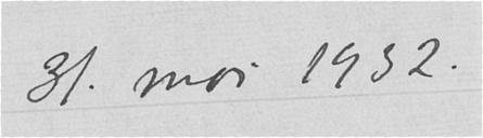31. mai 1932.
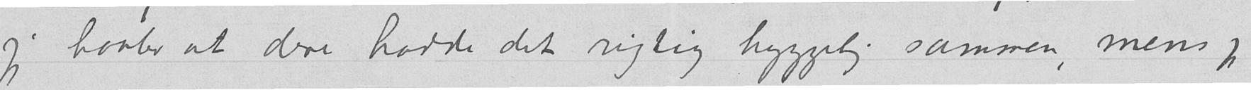jeg haaber at dere hadde det rigtig hyggelig sammen, mens jeg
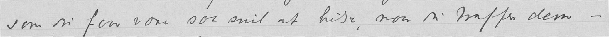som du faar være saa snil at hilse, naar du traffer dem –
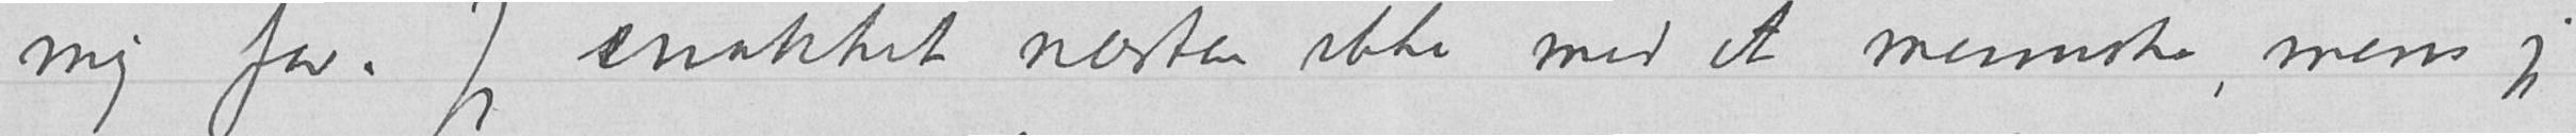mig for. Jeg snakket næsten ikke med et menneske, mens jeg
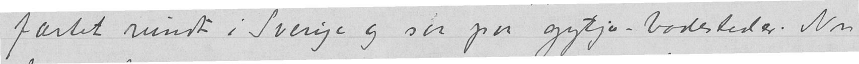fartet rundt i Sverige og saa paa gytje-badesteder. Nu
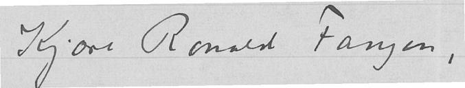Kjære Ronald Fangen,
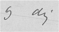og dig
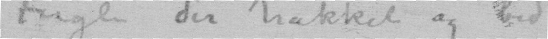Fugle der hakket og bed
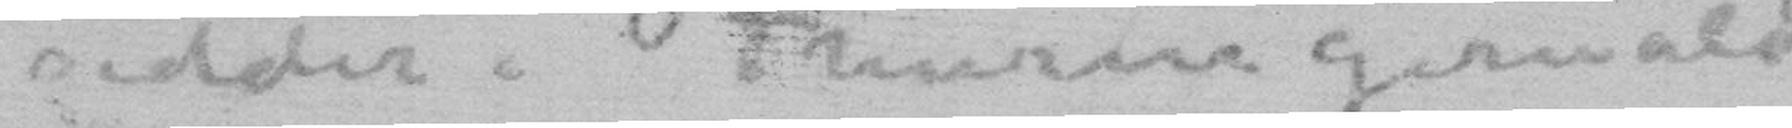sidder i Thüringerwald
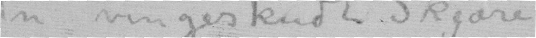En vingeskudt Skjære
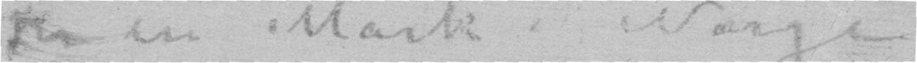på en Mark i Norge
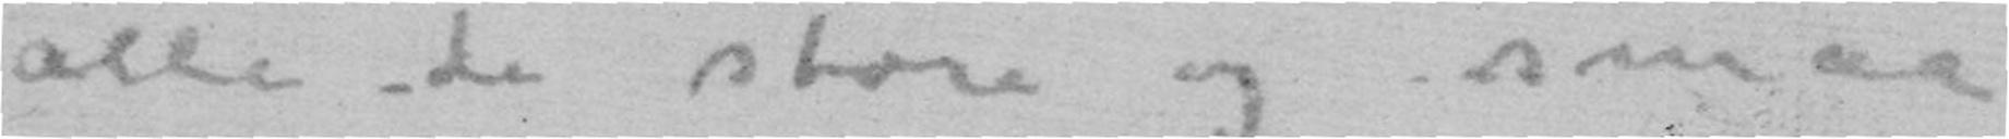alle de store og smaa
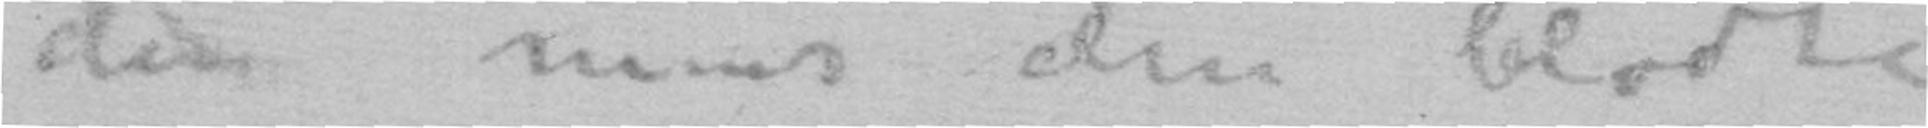den mens den blødte
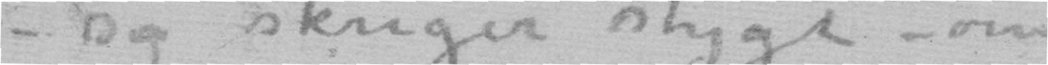– Og skriger stygt – om
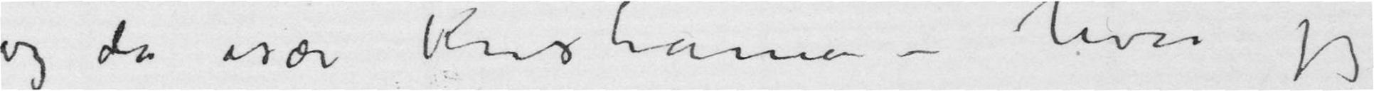og da især Kristiania – hvor jeg
